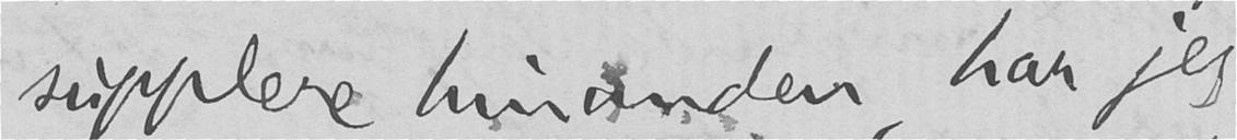supplere hinanden, har jeg
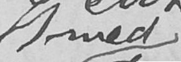med
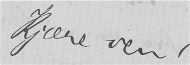Kjære ven!
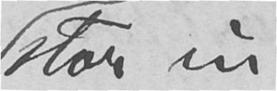stor in-
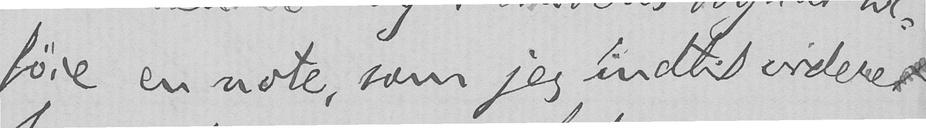føie en note, som jeg indtil videre
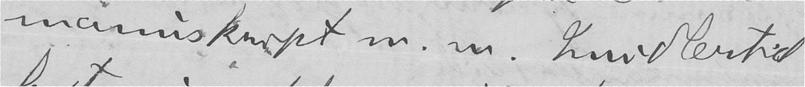manuskript m. m. Imidlertid
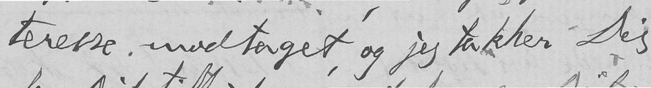teresse modtaget, og jeg takker Dig
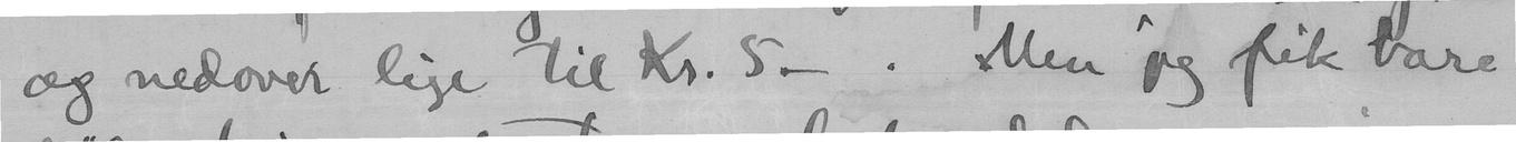og nedover lige til Kr. 5 -. Men jeg fik bare
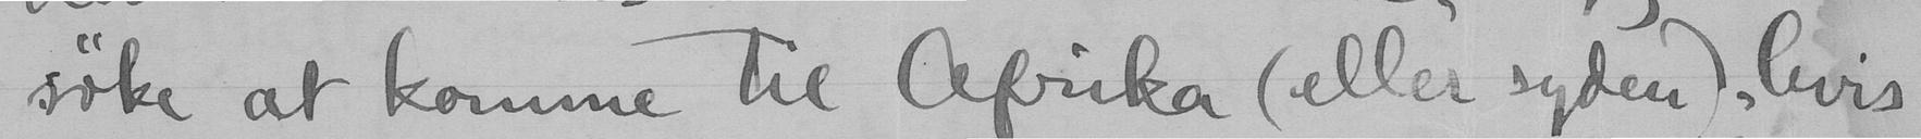søke at komme til Afrika (eller syden), hvis
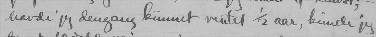havde jeg dengang kunnet ventet 1/2 aar, kunde jeg
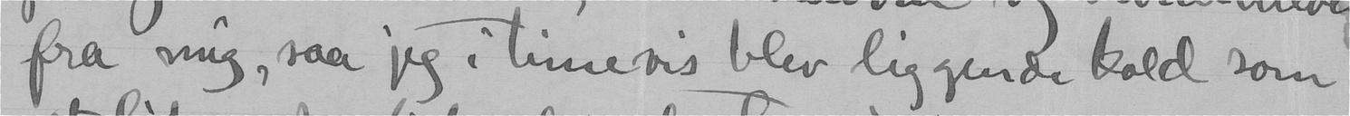fra mig, saa jeg i timevis blev liggende kold som
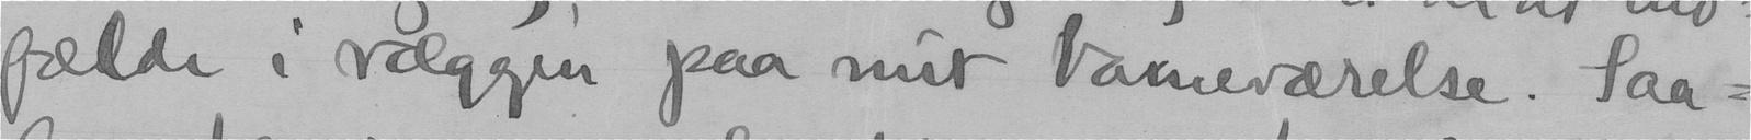fælde i væggen paa mit barneværelse. Saa-
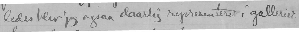ledes blev jeg ogsaa daarlig representeret i galleriet.
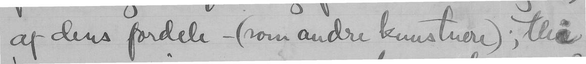af dens fordele - (som andre kunstnere); thi
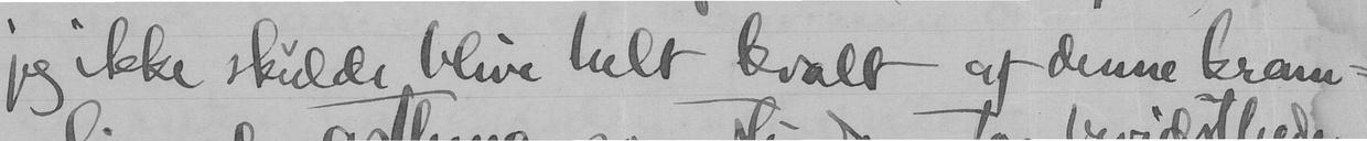jeg ikke skulde blive helt kvalt af denne kram-
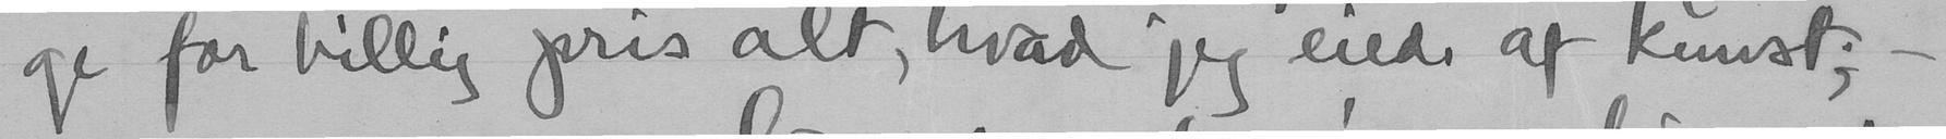ge for billig pris alt, hvad jeg eiede af kunst; -
